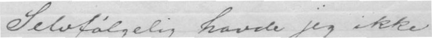Selvfølgelig havde jeg ikke
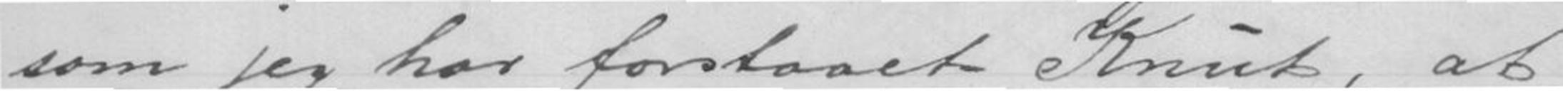som jeg har forstaaet Knut, at
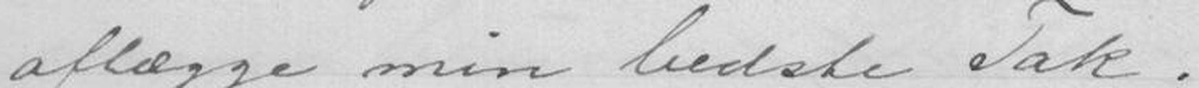aflægge min bedste Tak.
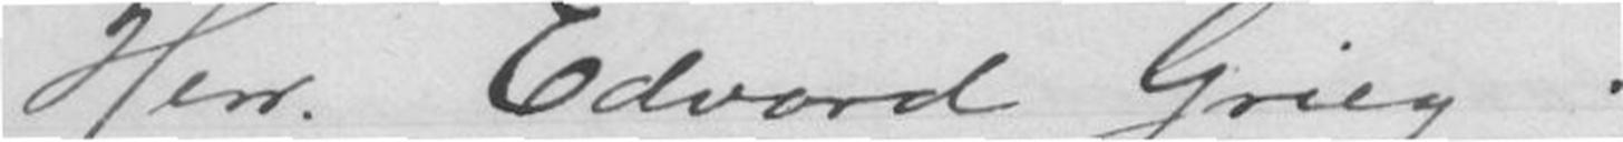Herr Edvard Grieg !
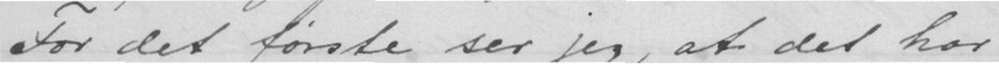For det første ser jeg, at det har
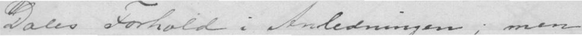Dales Forhold i Anledningen; men
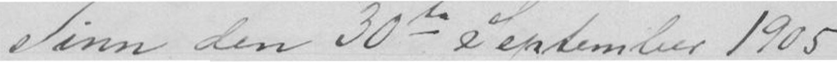Tinn den 30de September 1905
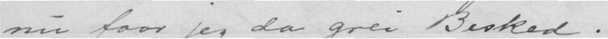nu faar jeg da grei Besked.
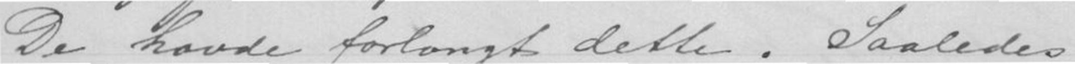De havde forlangt dette. Saaledes
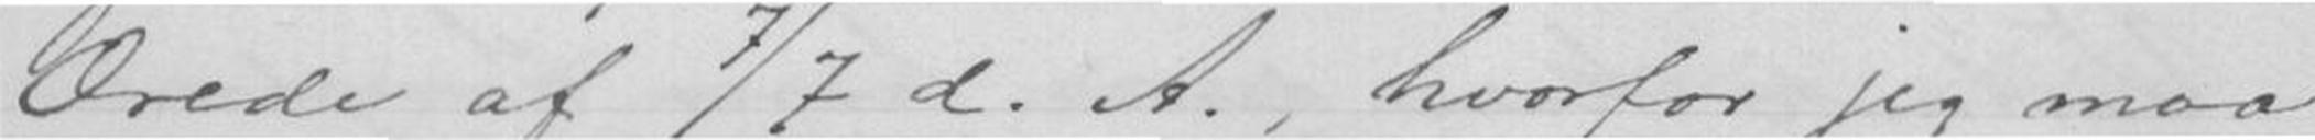Ærede af 7/7 d.A., hvorfor jeg maa
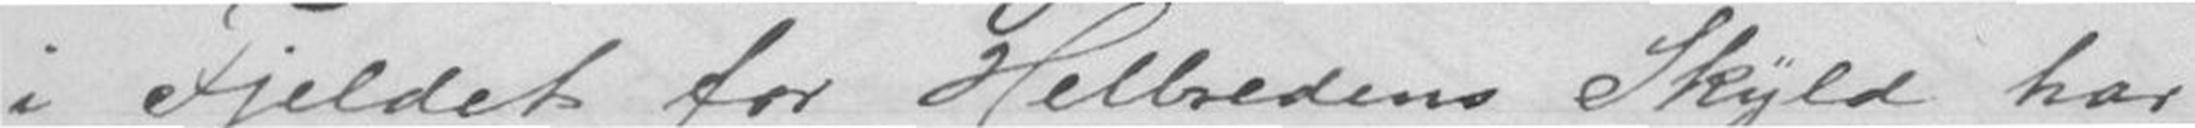i Fjeldet for Helbredens Skijld har
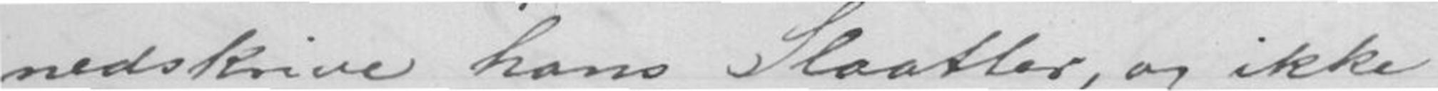nedskrive hans Slaatter, og ikke,
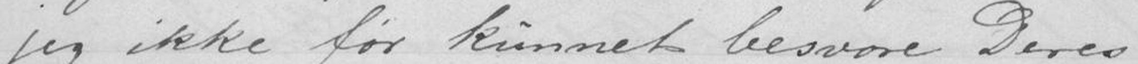jeg ikke för kunnet besvare Deres
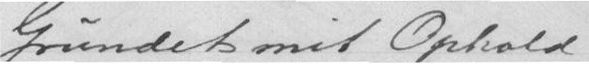Grundet mit Ophold
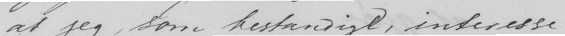at jeg, som bestandigt, interesse-
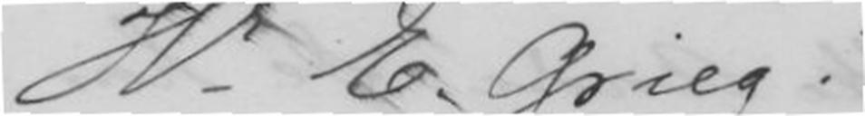Hr. E. Grieg!
